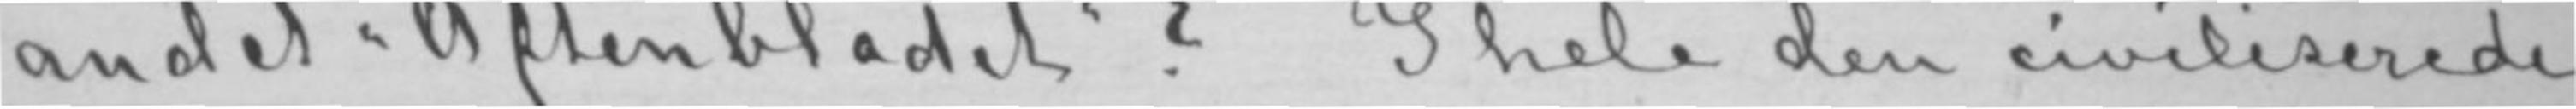andet «Aftenbladet»? I hele den civiliserede
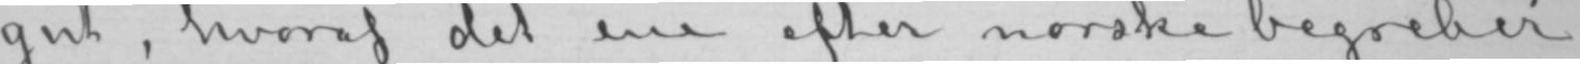gut», hvoraf det ene efter norske begreber
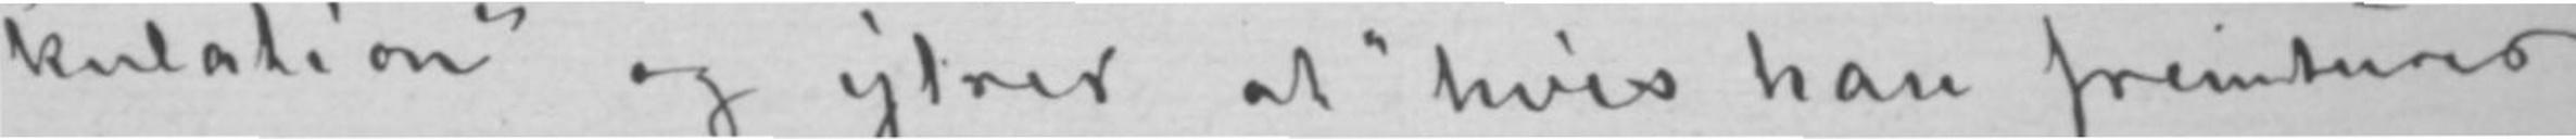kulation» og ytrer at «hvis han fremturer
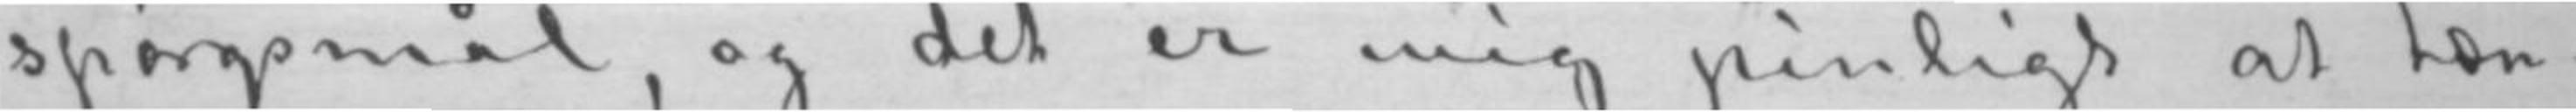spørgsmål, og det er mig pinligt at tæn-
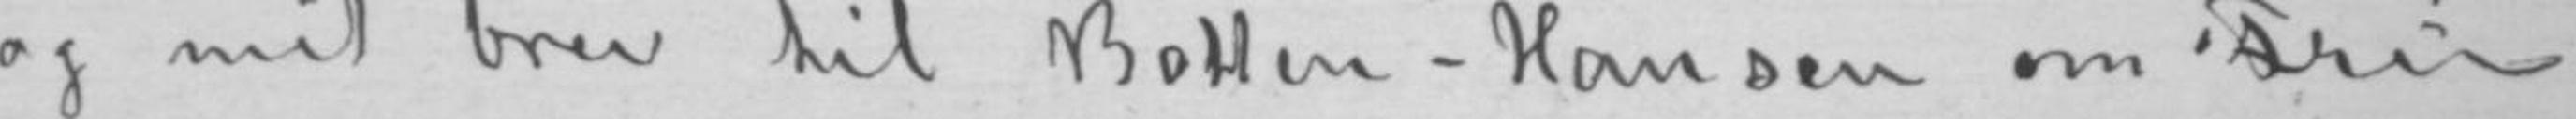og mit brev til Botten-Hansen om «Fru
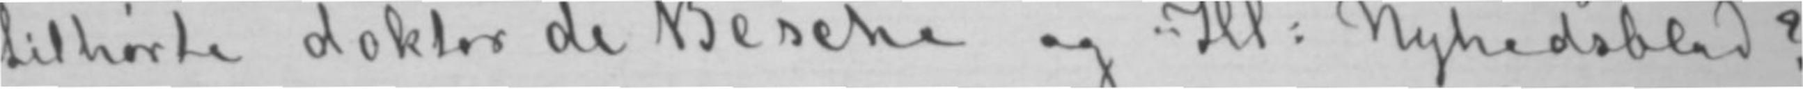tilhørte doktor de Besche og «Ill: Nyhedsblad?
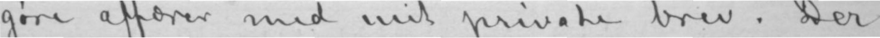gøre affærer med mit private brev. Der
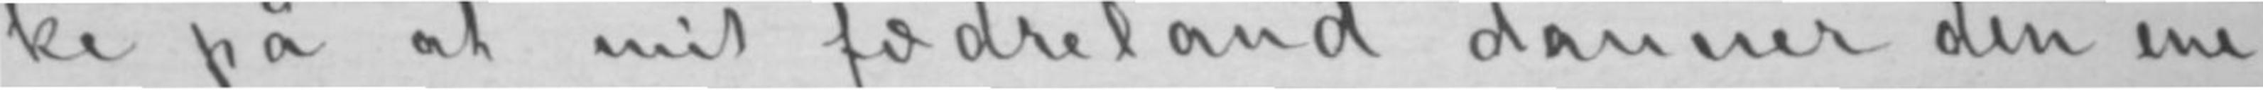ke på at mit fædreland danner den ene-
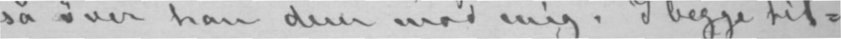så øver han dem mod mig. I begge til-
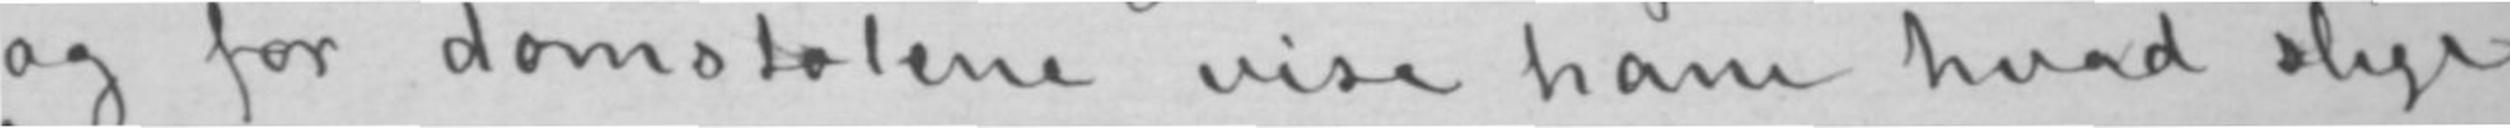og for domstolene vise ham hvad slige
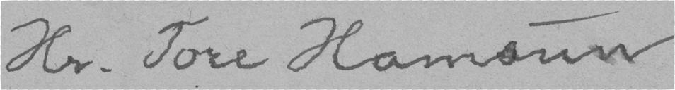Hr. Tore Hamsun
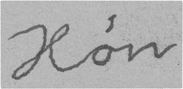Høn
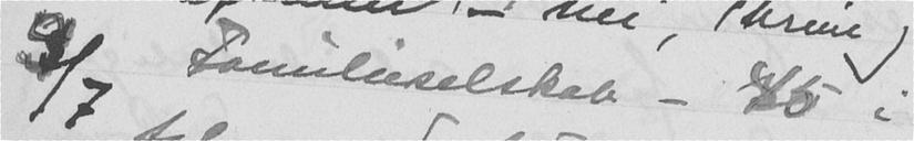3/7 Familieselskab - 45 i
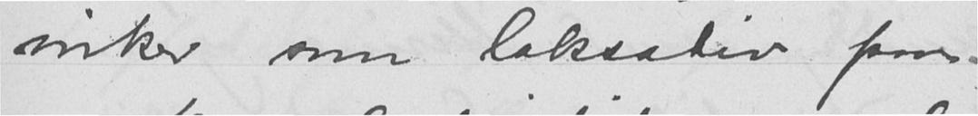virker som laksativ paa
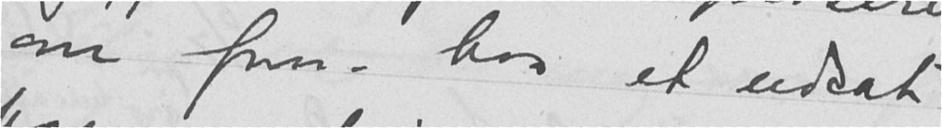om form. hos et udsat
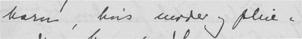barn, hvis moder og pleie-
